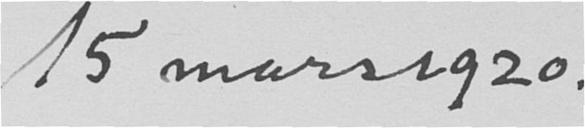15 mars 1920.
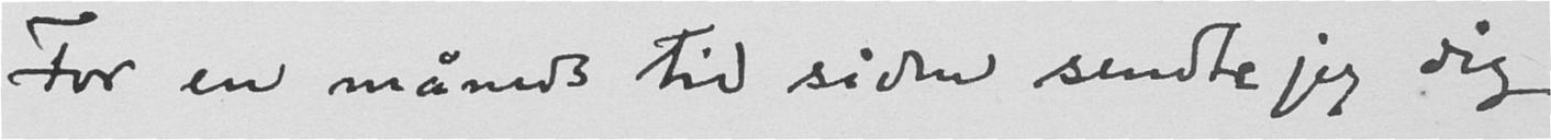For en måneds tid siden sendte jeg dig
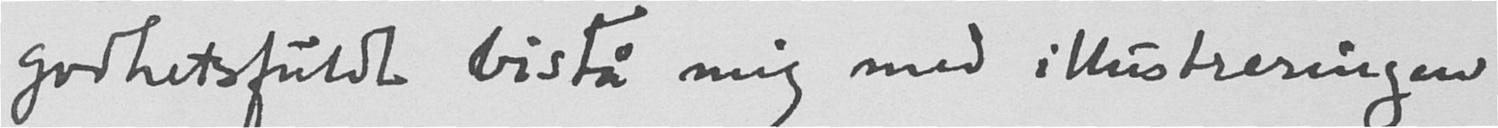godhetsfuldt bistå mig med illustreringen.
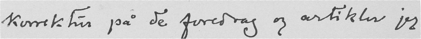korrektur på de foredrag og artikler jeg
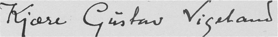Kjære Gustav Vigeland
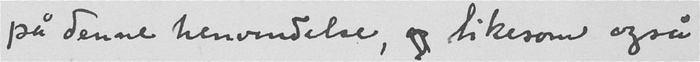på denne henvendelse, og likesom også
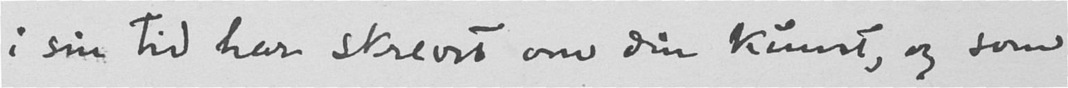i sin tid har skrevet om din kunst, og som
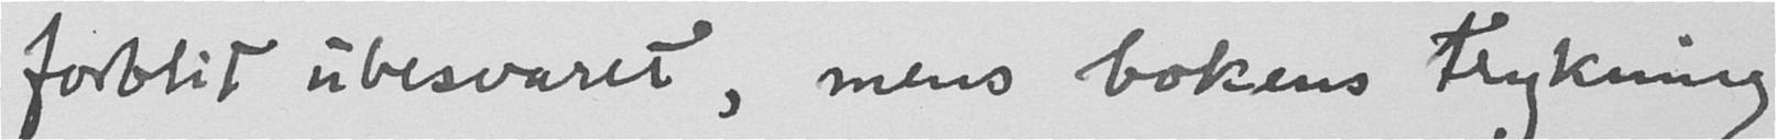forblit ubesvaret, mens bokens trykning
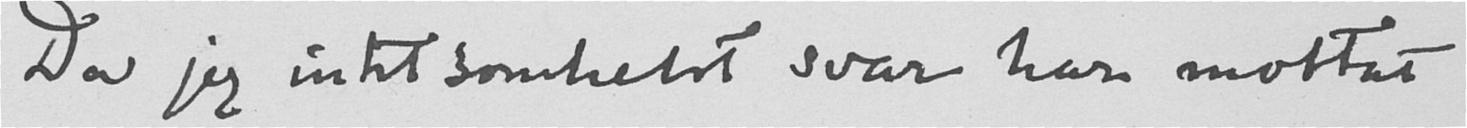Da jeg intet somhelst svar har mottat
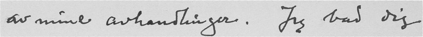av mine avhandlinger. Jeg bad dig
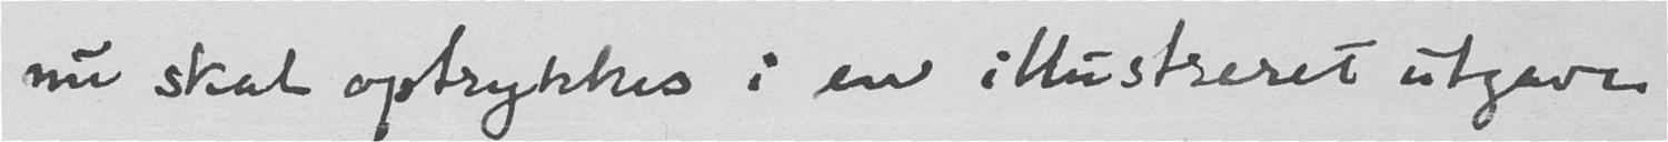nu skal optrykkes i en illustreret utgave
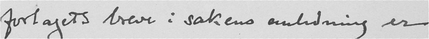forlagets breve i sakens anledning er
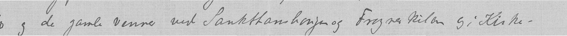Dito og de gamle venner ved Sankthanshaugen og Frognerkilen og i Kirke-
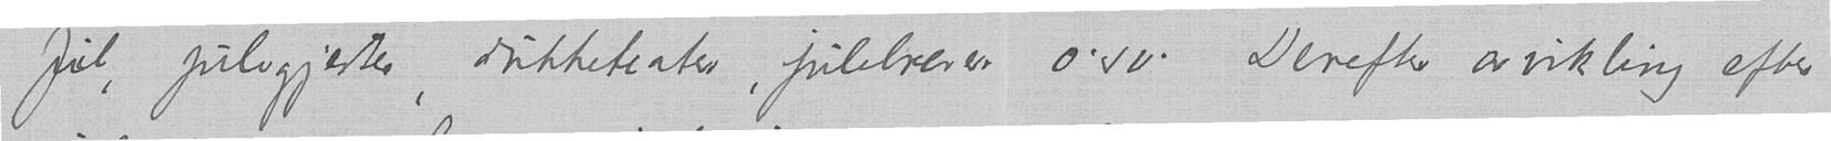Jul, julegjester, dukketeater, julebrever o.s.v. Derefter avvikling efter
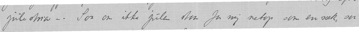julestria –. Saa om ikke julen staar for mig netop som en sæk, saa
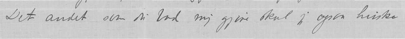Det andet som du bad mig gjøre skal jeg ogsaa huske
